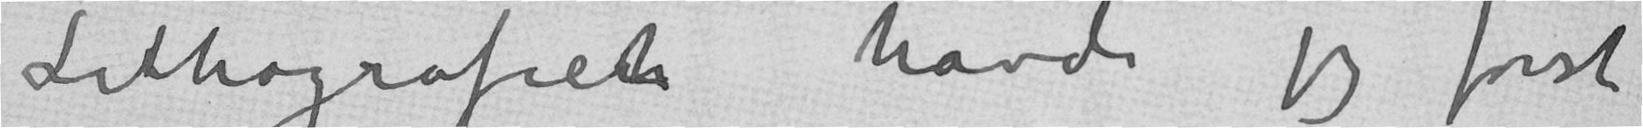Lithografiet havde jeg først
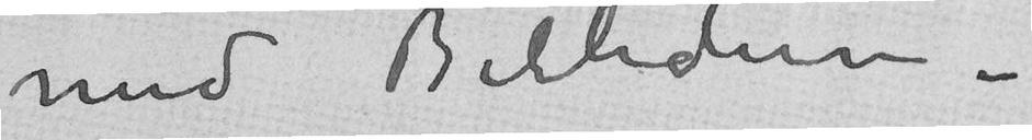med Billederne –
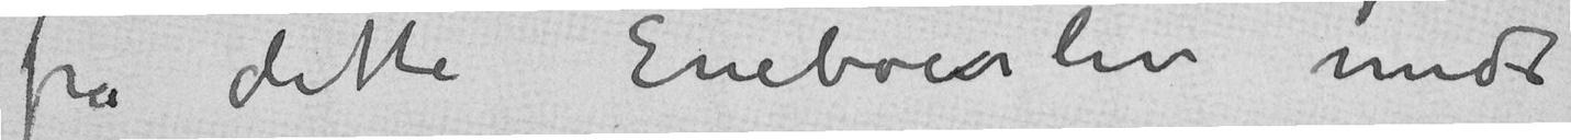fra dette Eneboerliv midt
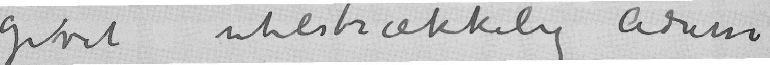givet utilstrækkelig Adresse
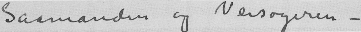Saamanden og Veisøgeren –
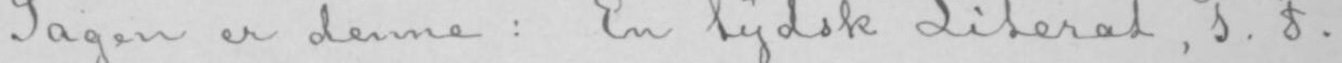Sagen er denne: En tydsk Literat, P. F.
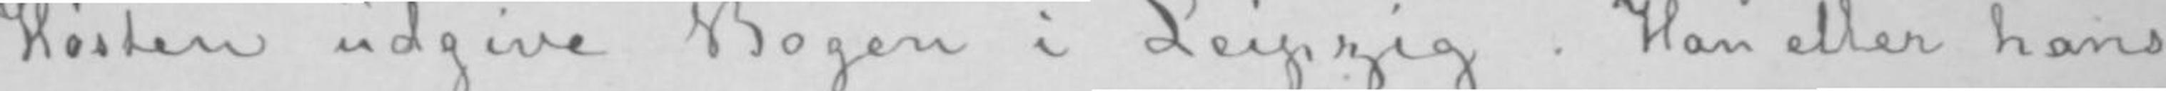Høsten udgive Bogen i Leipzig. Han eller hans
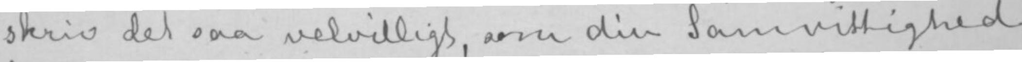skriv det saa velvilligt, som din Samvittighed
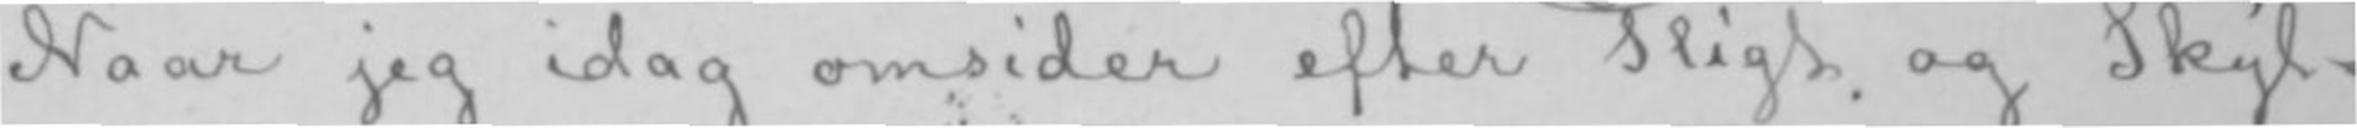Naar jeg idag omsider efter Pligt og Skyl-
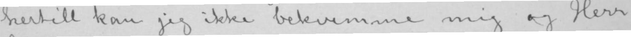hertill kan jeg ikke bekvemme mig og Herr
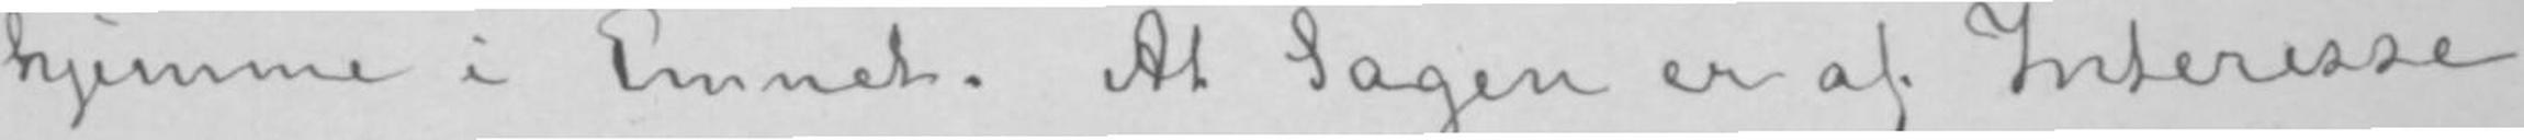hjemme i Emnet. At Sagen er af Interesse
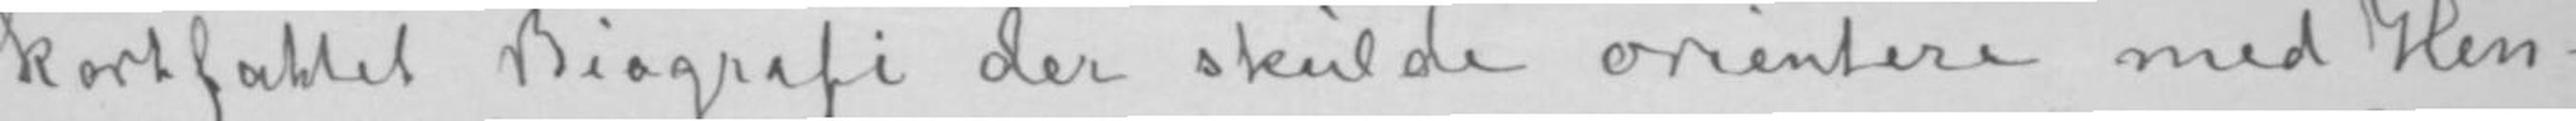kortfattet Biografi der skulde orientere med Hen-
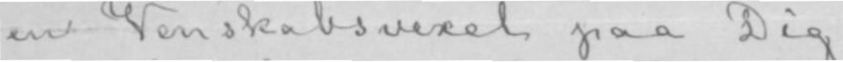en Venskabsvexel paa Dig.
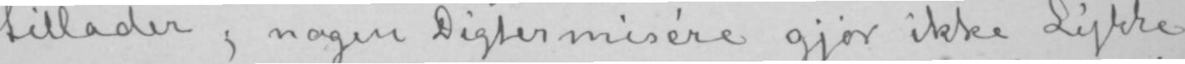tillader; nogen Digtermisère gjør ikke Lykke
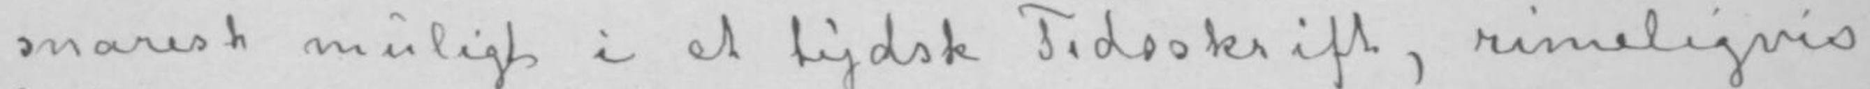snarest muligt i et tydsk Tidsskrift, rimeligvis
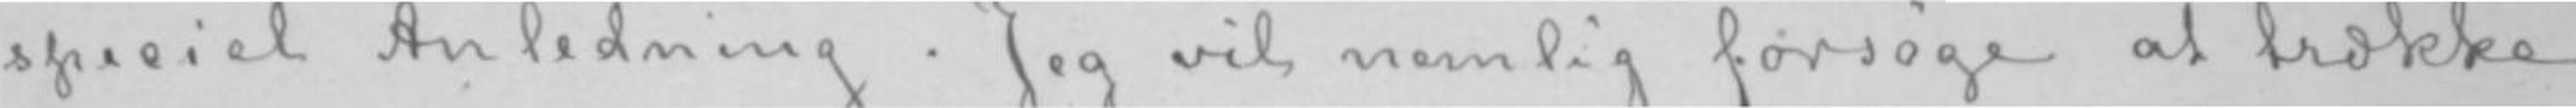speciel Anledning. Jeg vil nemlig forsøge at trække
